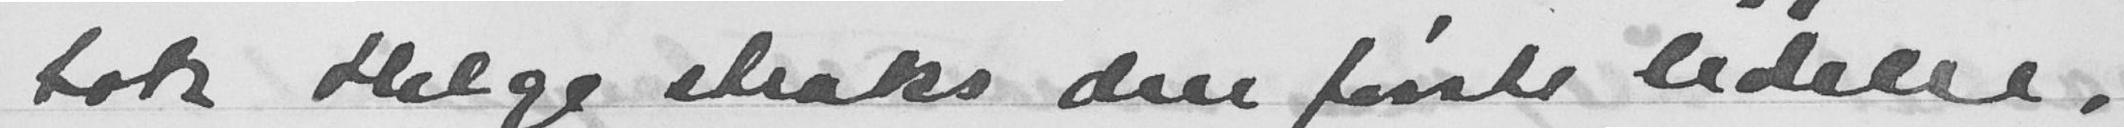tok Helge straks den første ledelse,
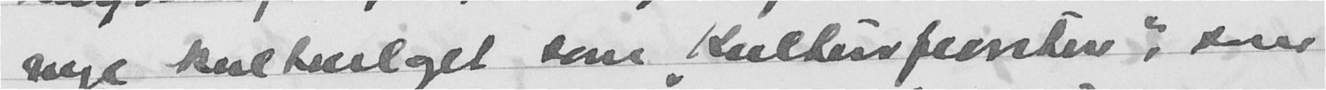nye kulturlaget som Kulturfevister, som
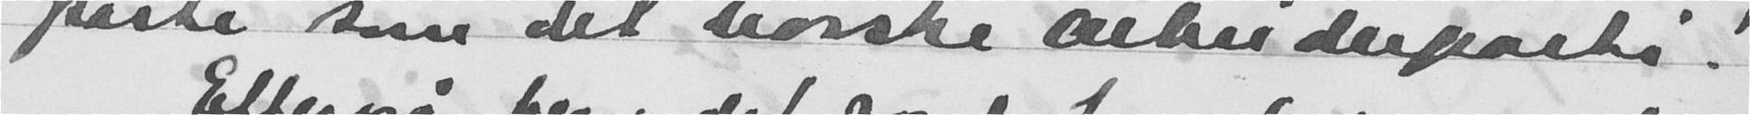parti som det norske arbeiderparti!
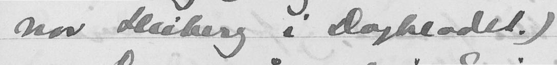nar Heiberg i Dagbladet.)
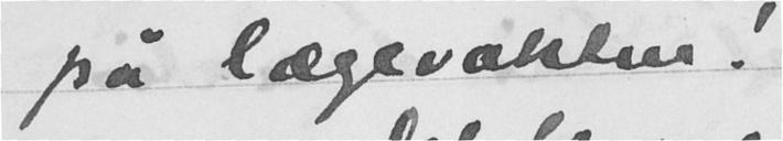på lægevakten!
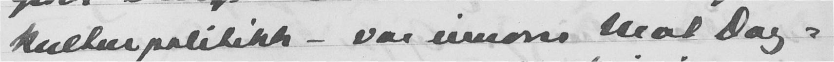kulturpolitikk - var innom Mot Dag-
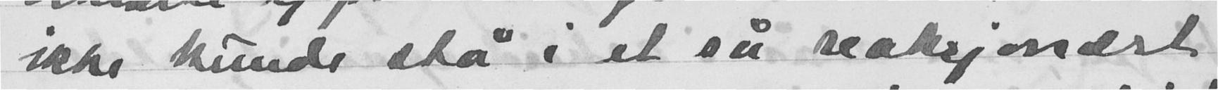ikke kunde stå i et så reaksjonært
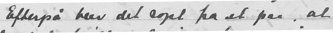Efterpå blev det ropt fra et par, at
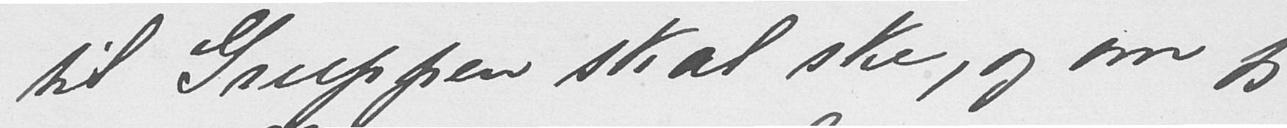til Gruppen skal ske, og om jeg
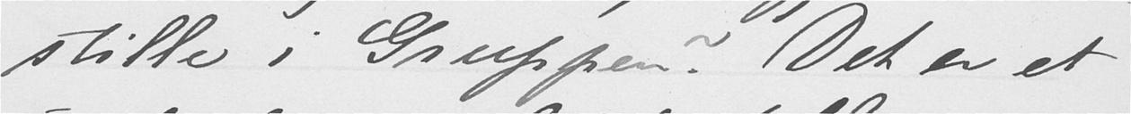stille i Gruppen? Det er et
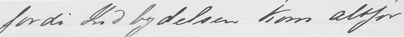fordi Indbydelsen kom altfor
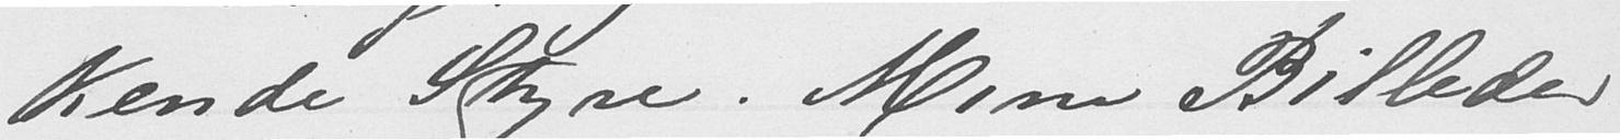kende Styre. Mine Billeder
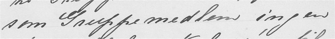som Gruppemedlem ingen
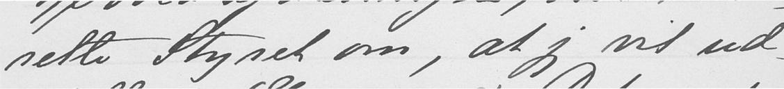rette Styret om, at jeg vil ud-
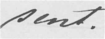sent.
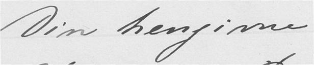Din hengivne
